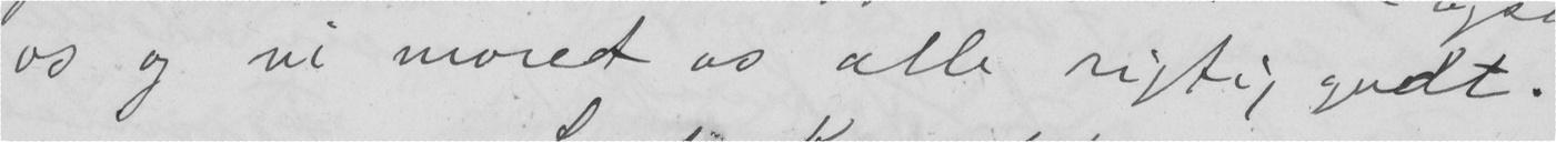os og vi moret os alle rigtig godt.
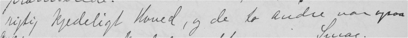rigtig kjedeligt Hoved, og de to andre var ogsaa
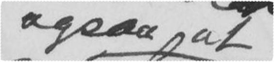ogsaa, at
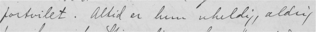fortvilet. Altid er hun uheldig, aldrig
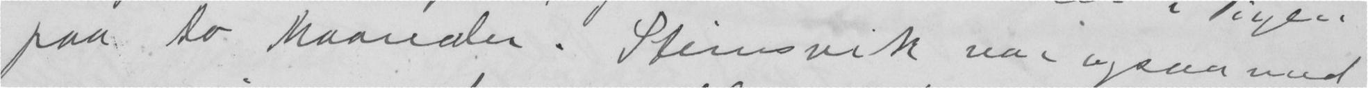paa to Maander. Steinsvik var ogsaa med
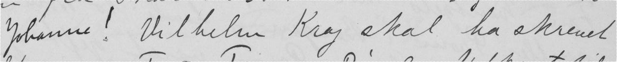Johanne! Vilhelm Krag skal ha skrevet
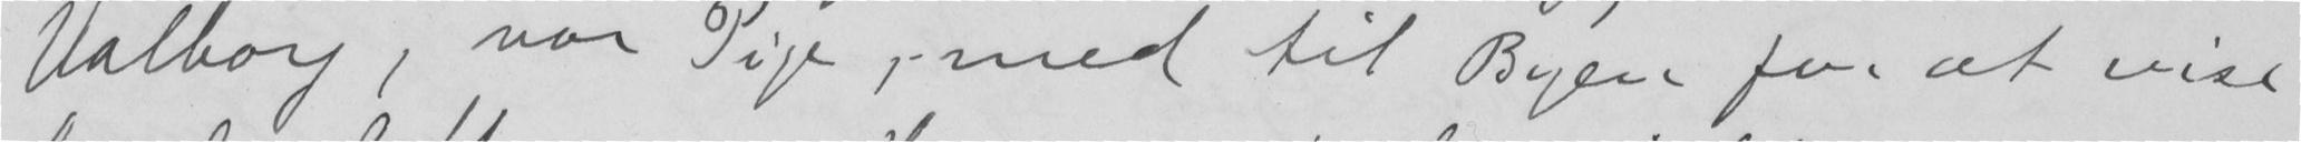Valborg, vor Pige, med til Byen for at vise
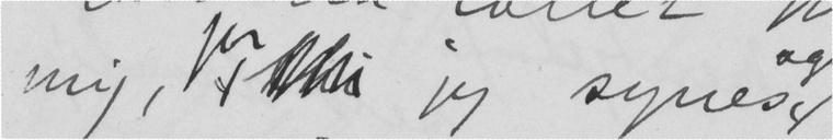mig,  jeg synes
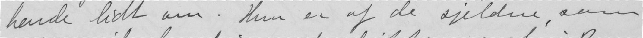hende lidt om. Hun er af de sjeldne som
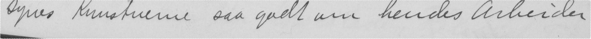synes Kunstnerne saa godt om hendes Arbeider
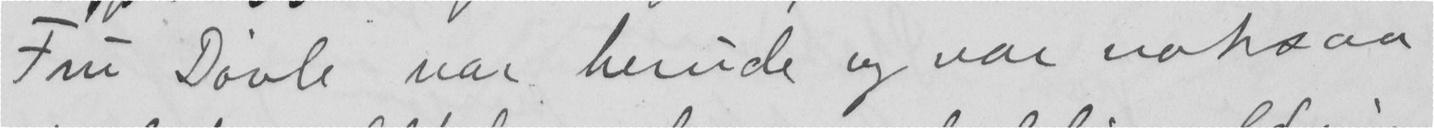Fru Døvle var herude og var noksaa
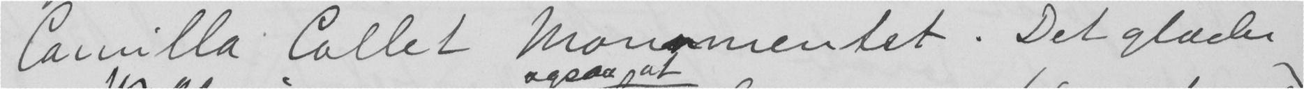Camilla Collet Monumentet. Det glæder
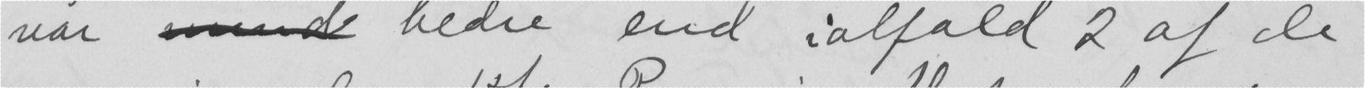var  bedre end ialfald 2 af de
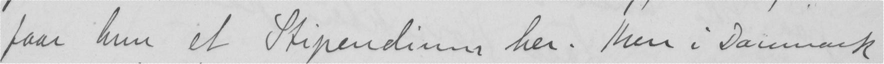faar hun et Stipendium her. Men i Danmark
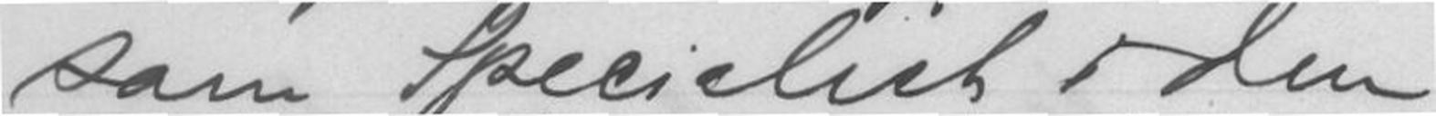som Specialist i den
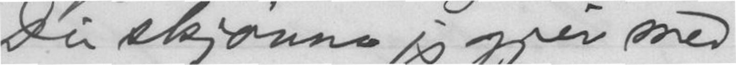Du skjønne jeg gjør med
